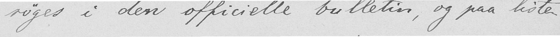søges i den officielle bulletin, og paa listen
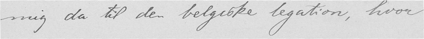mig da til den belgiske legation, hvor
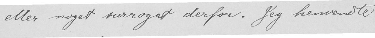eller noget surrogat derfor. Jeg henvendte
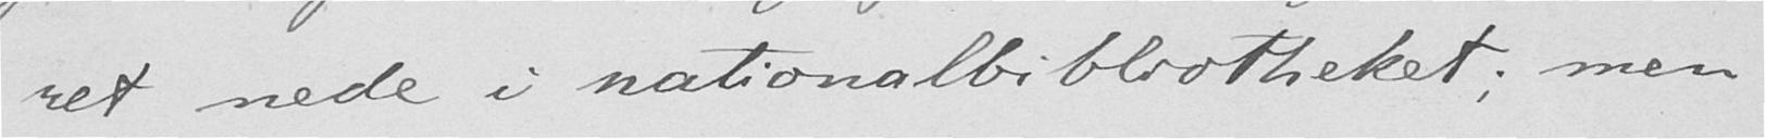ret nede i nationalbibliotheket; men
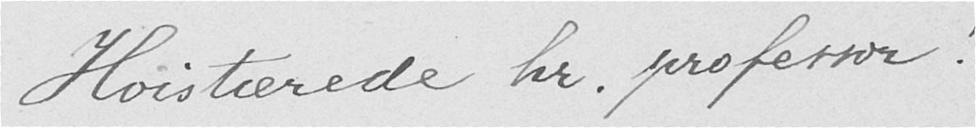Høistærede hr. professor!
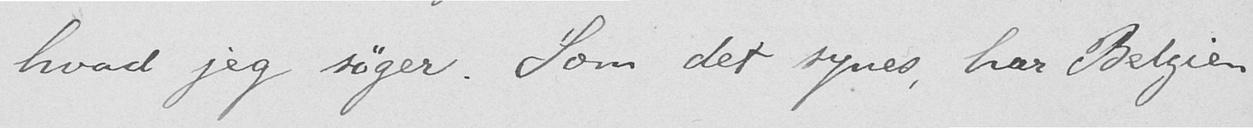hvad jeg søger. Som det synes, har Belgien
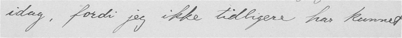idag, fordi jeg ikke tidligere har kunnet
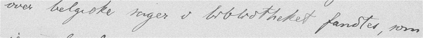over belgiske sager i bibliotheket fandtes, som
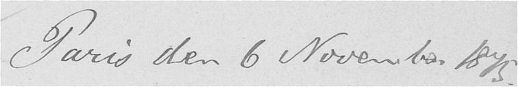Paris den 6 November 1875.
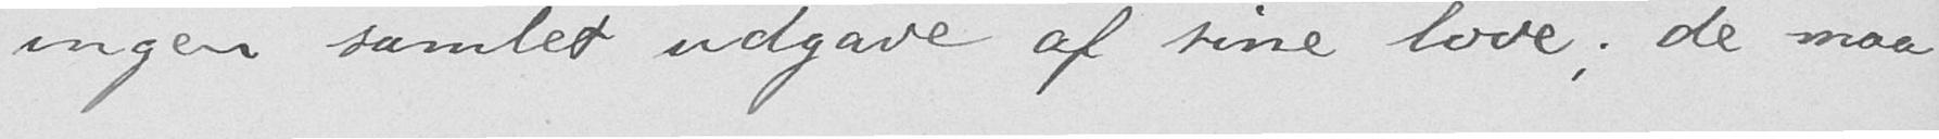ingen samlet udgave af sine love; de maa
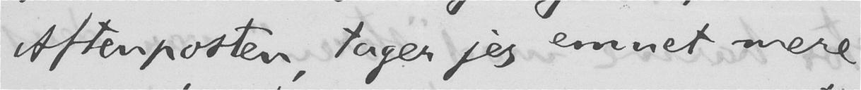Aftenposten, tager jeg emnet mere
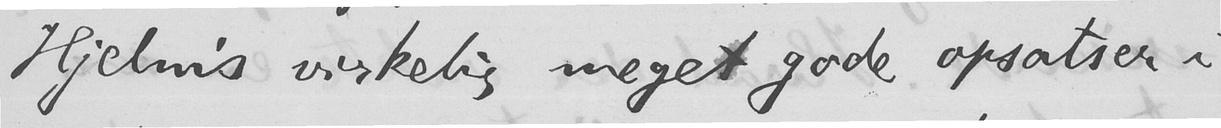Hjelm's virkelig meget gode opsatser i
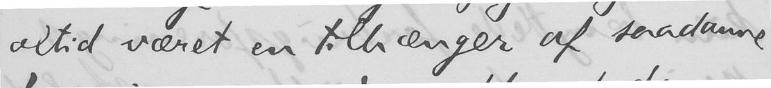altid været en tilhænger af saadanne
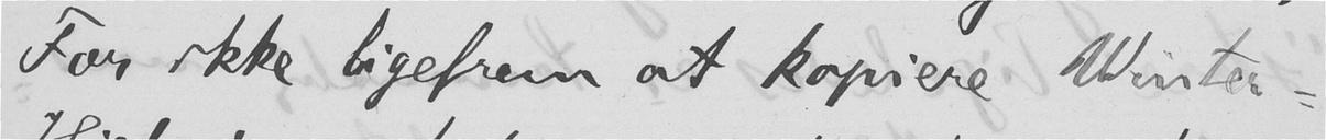For ikke ligefrem at kopiere Winter-
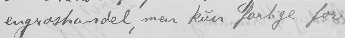engroshandel, men kun farlige for
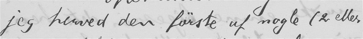jeg herved den første af nogle (2 eller
